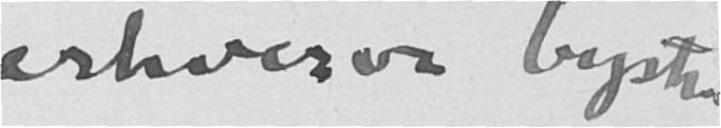erhverve bysten
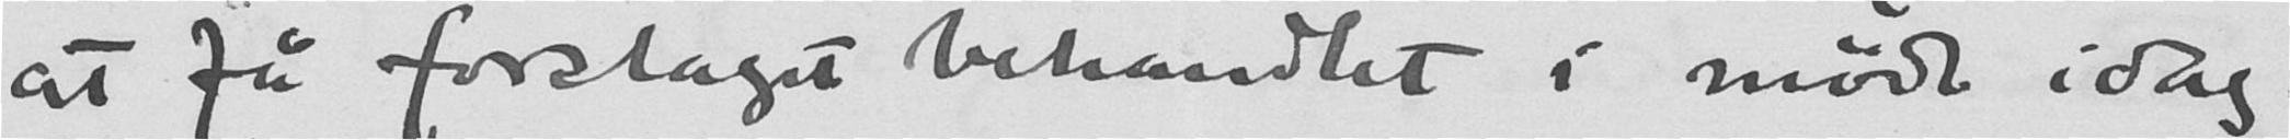at få forslaget behandlet i møde idag.
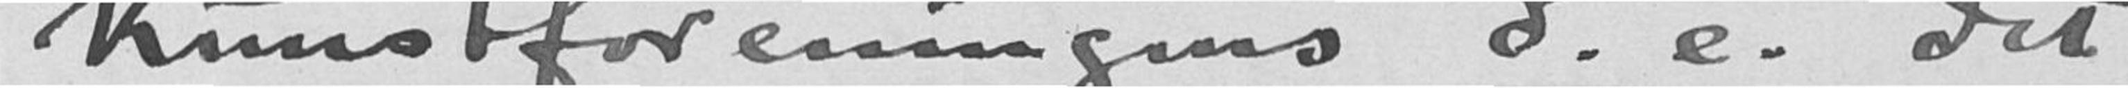Kunstforeningens d.e. det
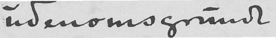udenomsgrunde.
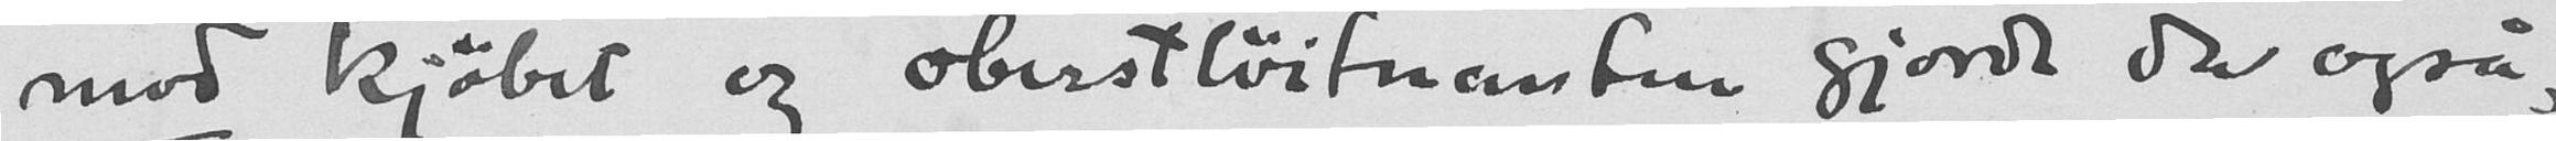mod kjøbet og oberstløitnanten gjorde da også,
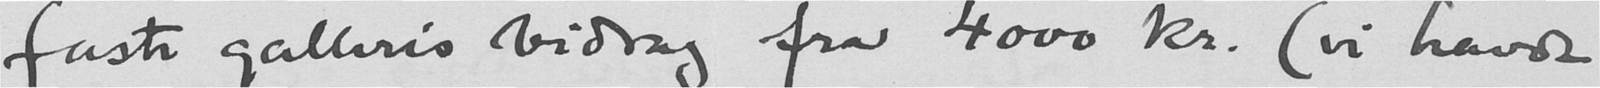faste galleris bidrag fra 4000 kr. (vi havde
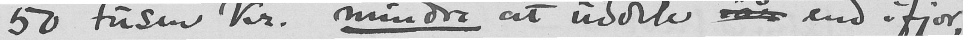50 tusen Kr. mindre at uddele iår end ifjor,
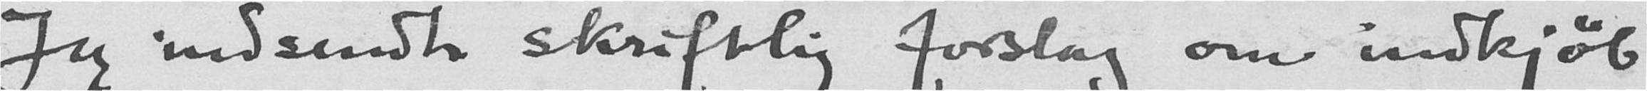Jeg indsendte skriftlig forslag om indkjøb
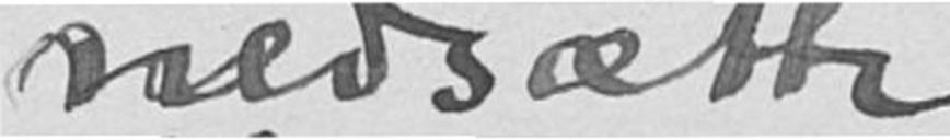nedsætte
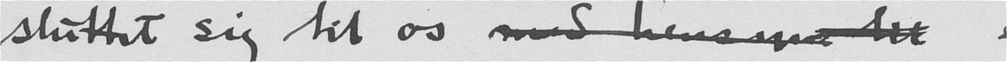sluttet sig til os med hensyn til
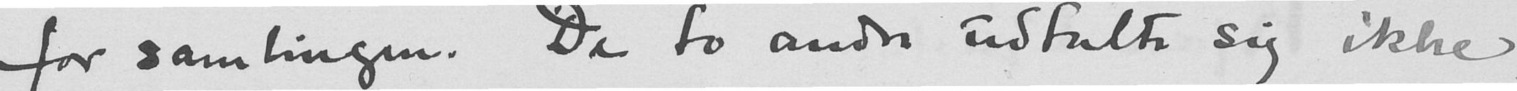for samlingen. De to andre udtalte sig ikke
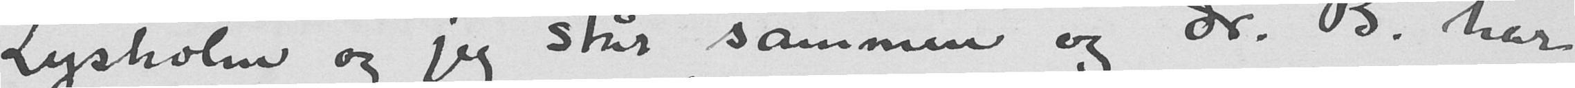Lysholm og jeg står sammen og dr. B. har
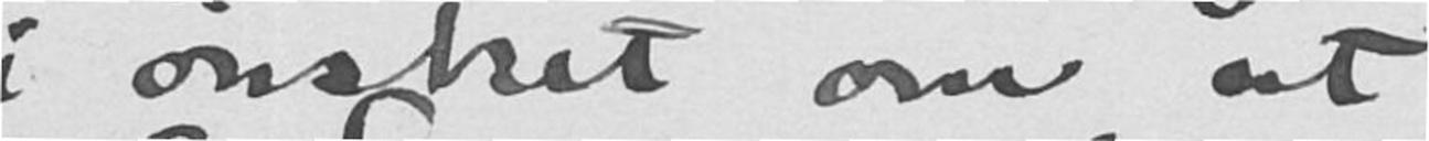i ønsket om at
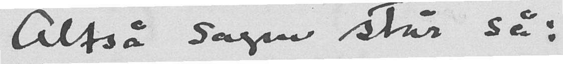Altså sagen står så:
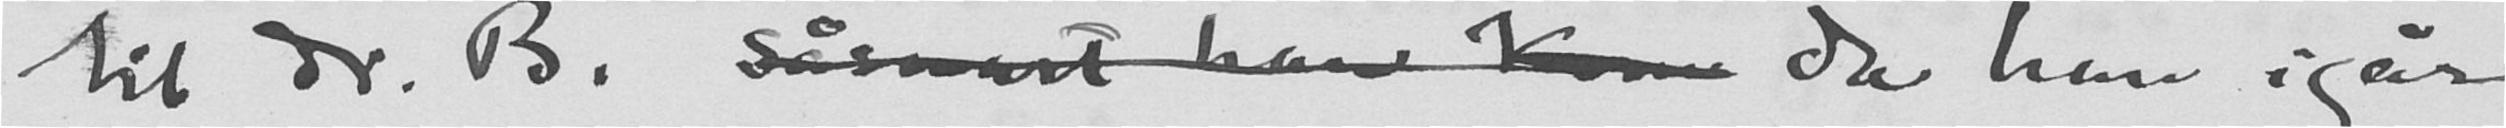til dr. B. såsnart han kom da han igår
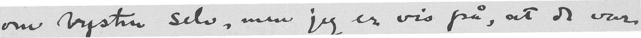om bysten selv, men jeg er vis på, at de var
